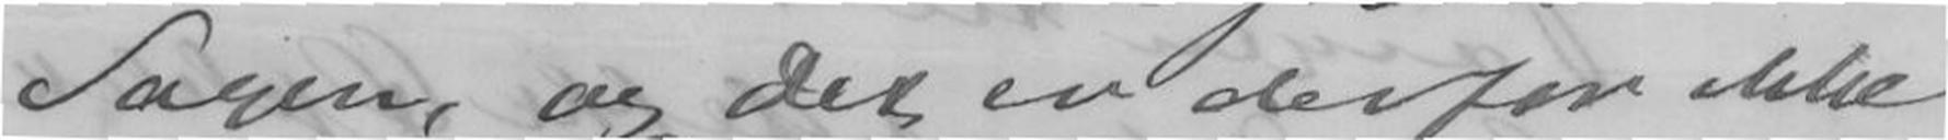Sagen, og det er derfor ikke
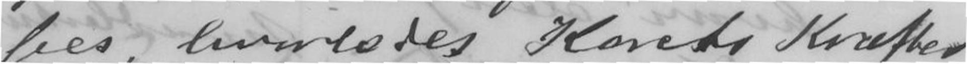sees, hvorledes Korets Kræfter
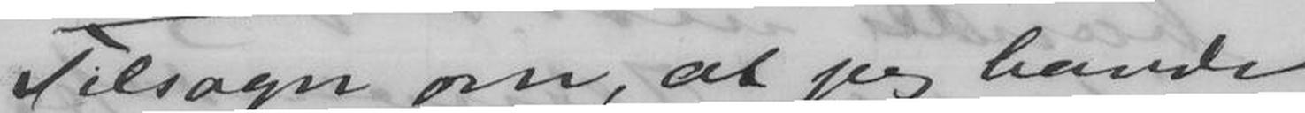Tilsagn om, at jeg havde
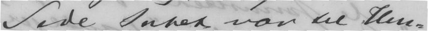Side Intet var til Hin-
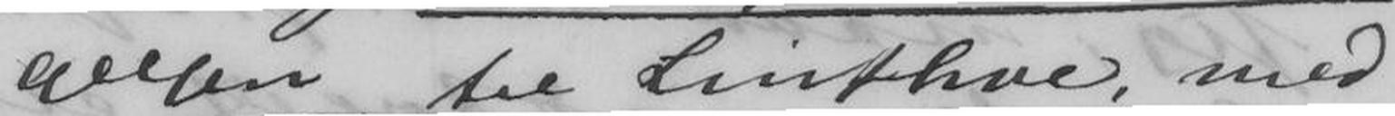gelsen til Linthve, med
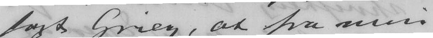sagt Grieg, at fra min
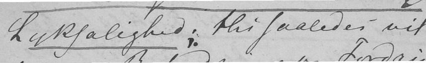Lyksalighed; thi saaledes vil
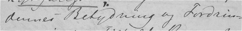dennes Betydning og Fordrin-
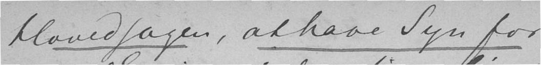hovedsagen, at have Syn for
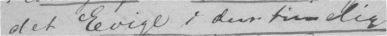det Evige i den timelig
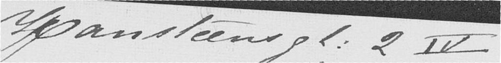Hansteens gt: 2 IV
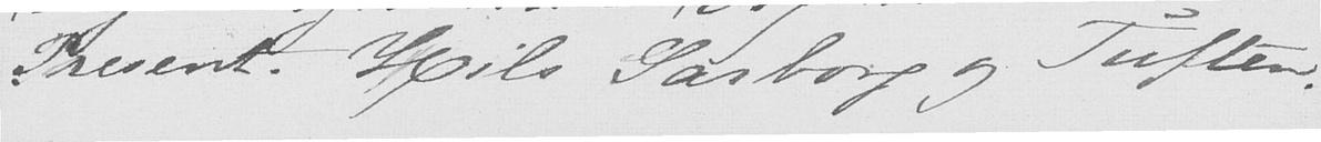Present. Hils Garborg og Tuften.
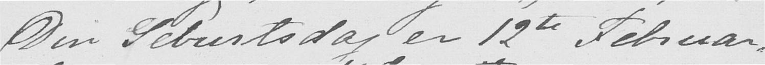Din Geburtsdag er 12te Februar.
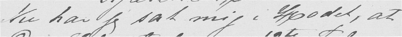Nu har jeg sat mig i Hodet, at
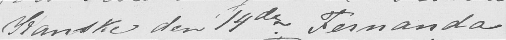Kanske den 14de. Fernanda
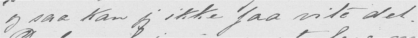og saa kan jeg ikke faa vite det.
